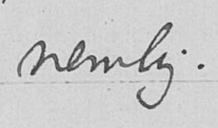nemlig.
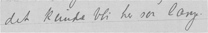det kunde bli her saa længe.
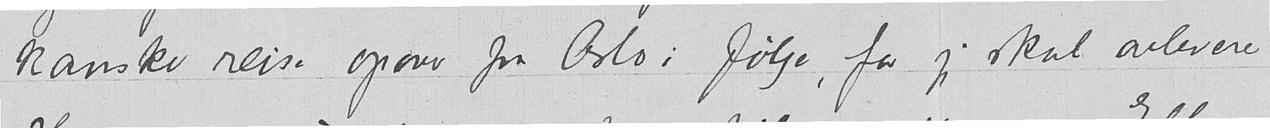kanske reise opover fra Oslo i følge, for jeg skal avlevere
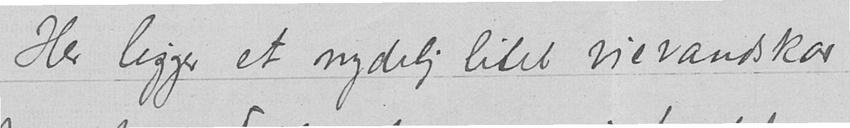Her ligger et nydelig litet vievandskar
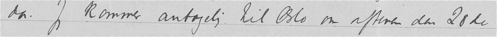da. Jeg kommer antagelig til Oslo om aftenen den 28de
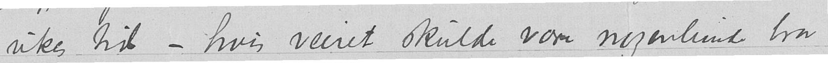ukes tid – hvis veiret skulde være nogenlunde bra
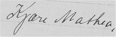Kjære Mathea,
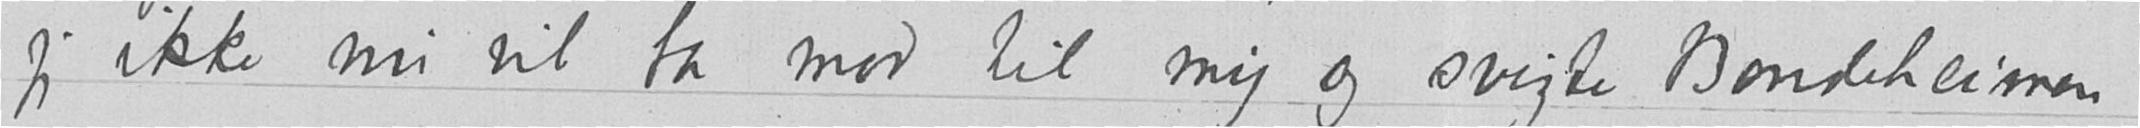jeg ikke nu vil ta mod til mig og svigte Bondeheimen
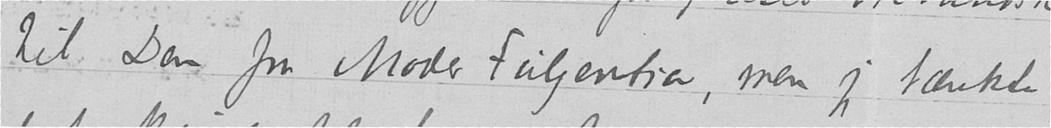til Dem fra Moder Fuljentia, men jeg tænkte
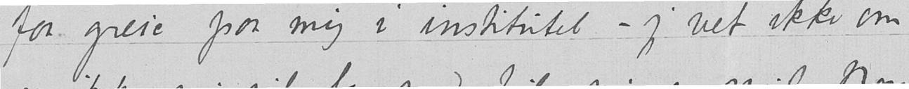faa greie paa mig i institutet – jeg vet ikke om
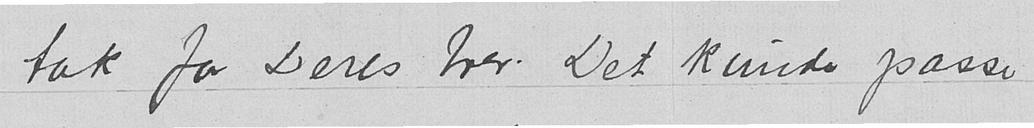tak for Deres brev. Det kunde passe
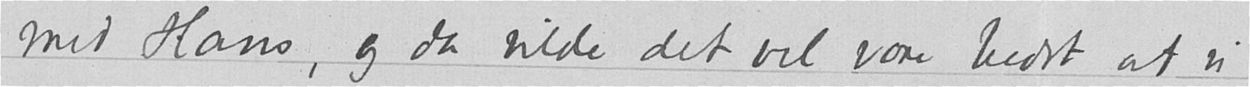med Hans, og da vilde det vel være bedst at vi
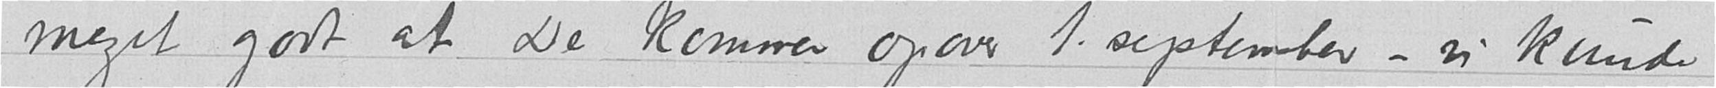meget godt at De kommer opover 1. september – vi kunde
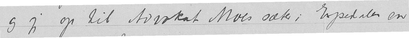og jeg op til Advokat Moes sæter i Espedalen en
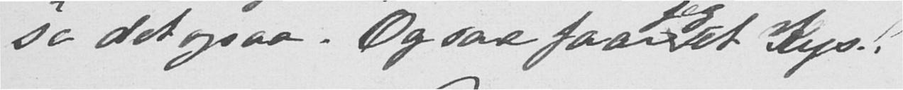se det ogsaa. Og saa faar et Kys!
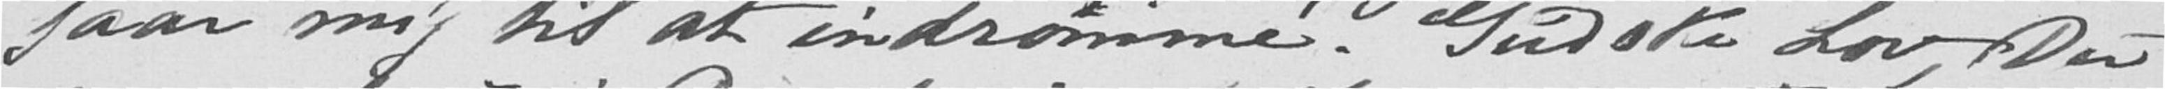faar mig til at indrømme. Gud ske Lov, Du
